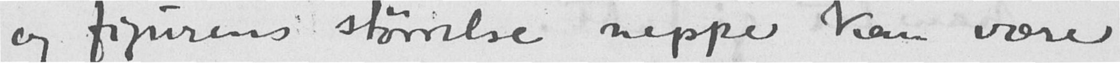og figurens størrelse neppe kan være
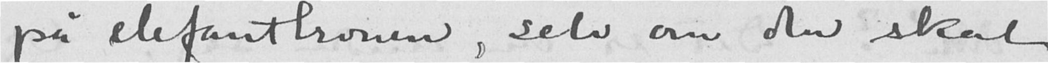på elefanttronen, selv om den skal
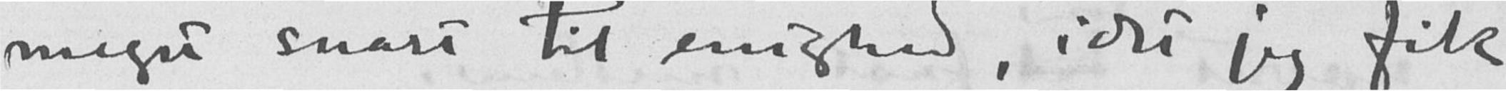meget snart til enighed, idet jeg fik
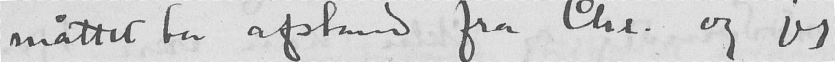måttet ta afstand fra Chr. og jeg
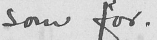som før.
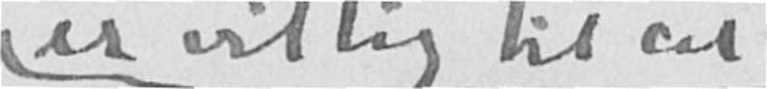er villig til at
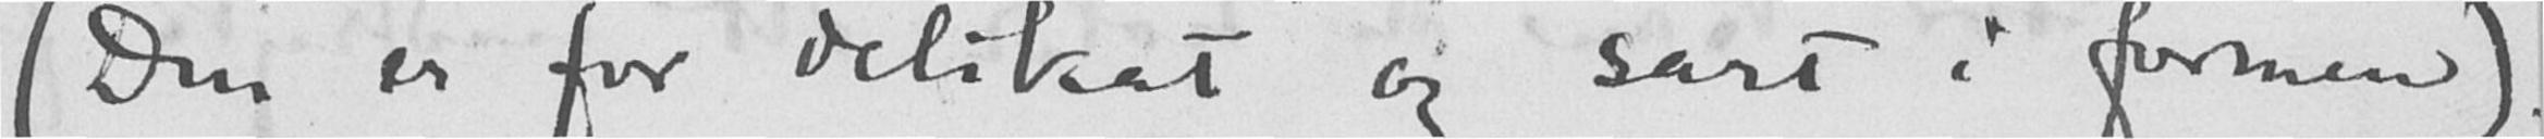(Den er for delikat og sart i formen).
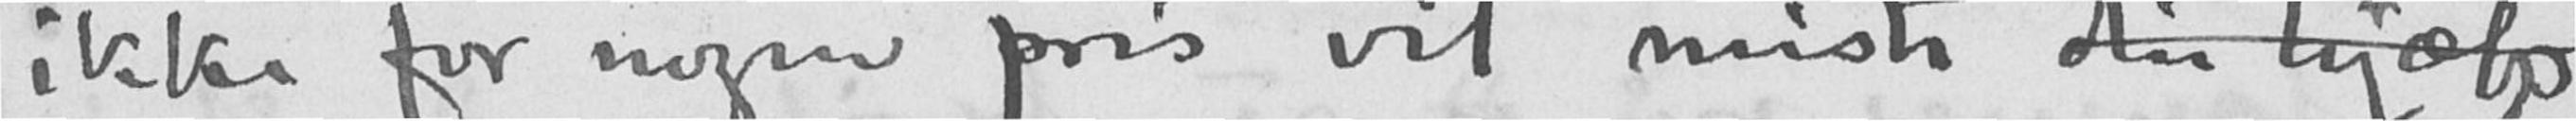ikke for nogen pris vil miste din hjælp
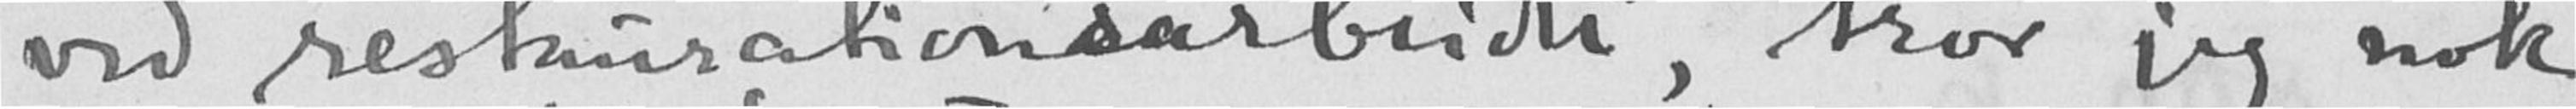ved restaurationsarbeidet, tror jeg nok
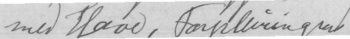med Have, Forpleiningens
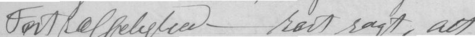Fortræffelighed - kort sagt, alt
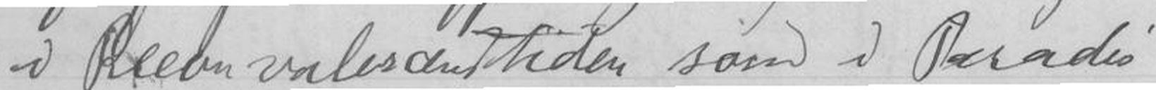i Reconvalesænstiden som i Paradis
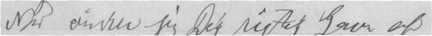Nu ønsker jeg Dig rigtig Gavn af
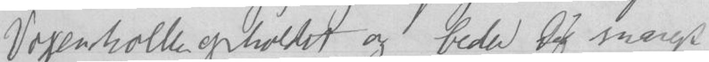Voxenkollenopholdet og beder Dig snarest
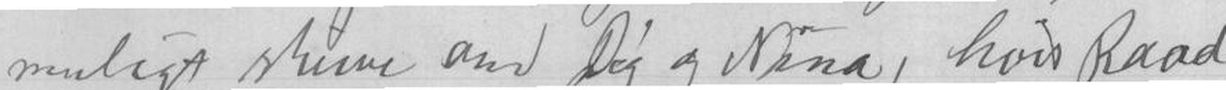muligt skrive om Dig og Nina, hvis Raad
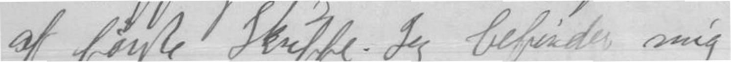af første Skuffe. Jeg befinder mig
